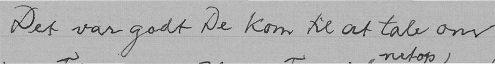Det var godt De kom til at tale om
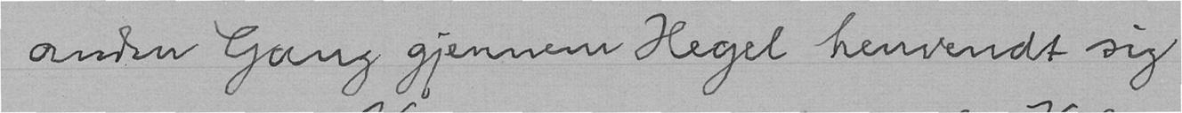anden Gang gjennem Hegel henvendt sig
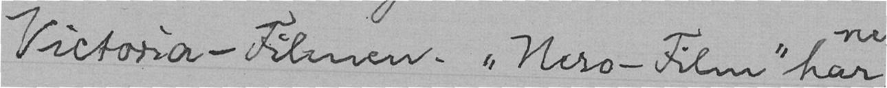Victoria-Filmen. "Nero-Film" har
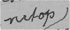netop
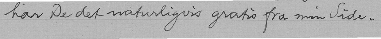har De det naturligvis gratis fra min Side.
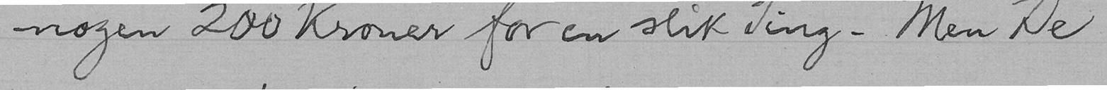nogen 200 Kroner for en slik Ting. Men De
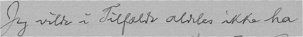Jeg vilde i Tilfælde aldeles ikke ha
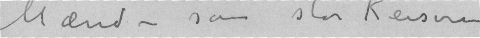Mænd – som stod Keiseren
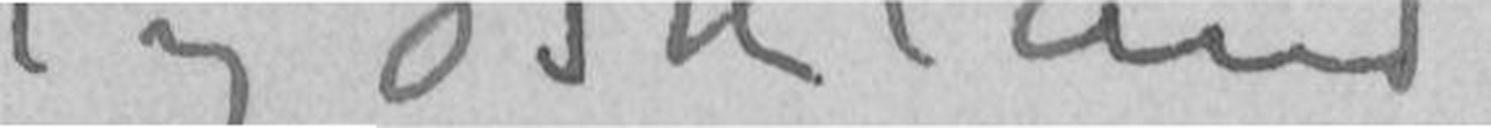Tydskland
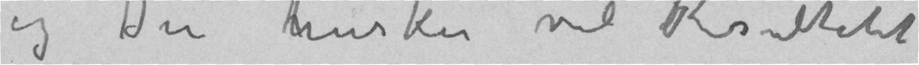og Du husker vel Resultatet
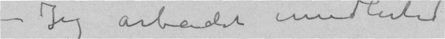– Jeg arbeidet imidlertid
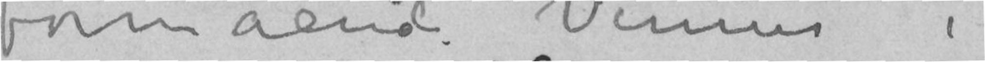formående Venner i
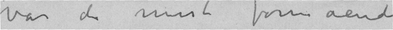var de mest formående
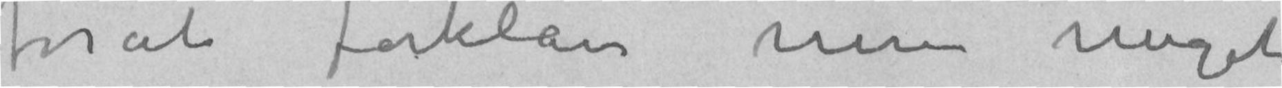forat forklare mine meget
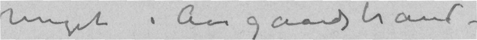meget i Aasgaardstrand –
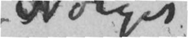Norges
